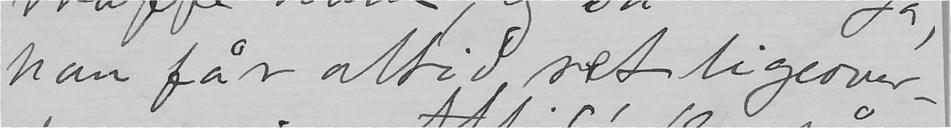han får altid ret ligeover-
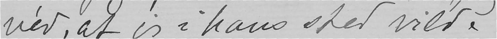véd, at jeg i hans sted vilde
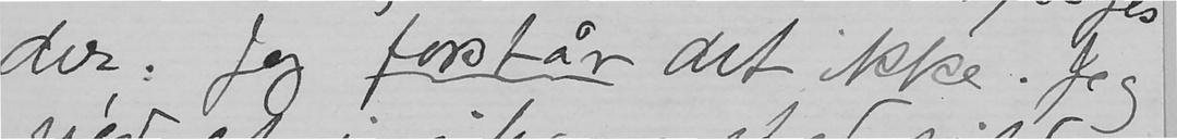der. Jeg forstår det ikke. Jeg
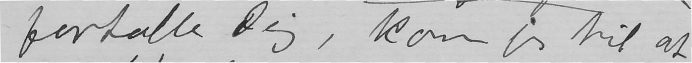fortælle Dig, kom jeg til at
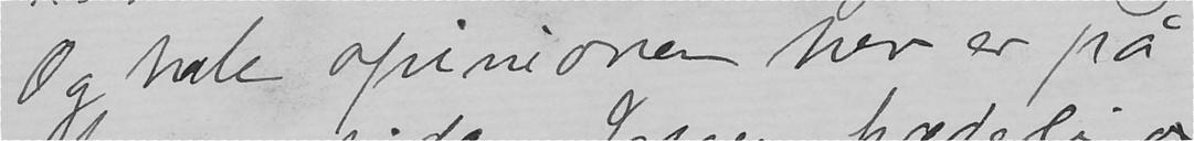Og hele opinionen her er på
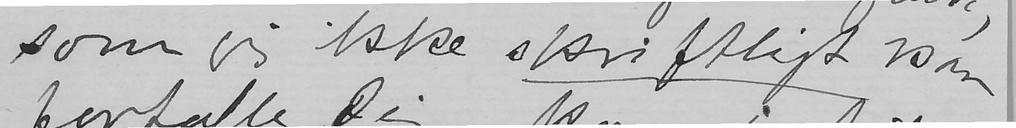som jeg ikke skriftligt kan
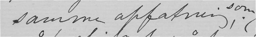samme opfatning,
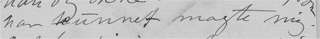han kunnet magte mig.
